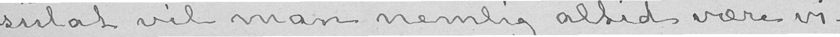sulat vil man nemlig altid være vi-
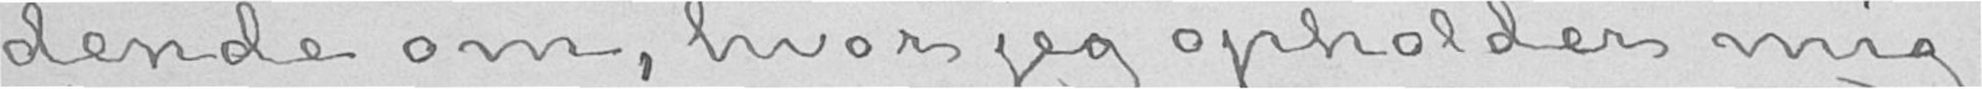dende om, hvor jeg opholder mig.
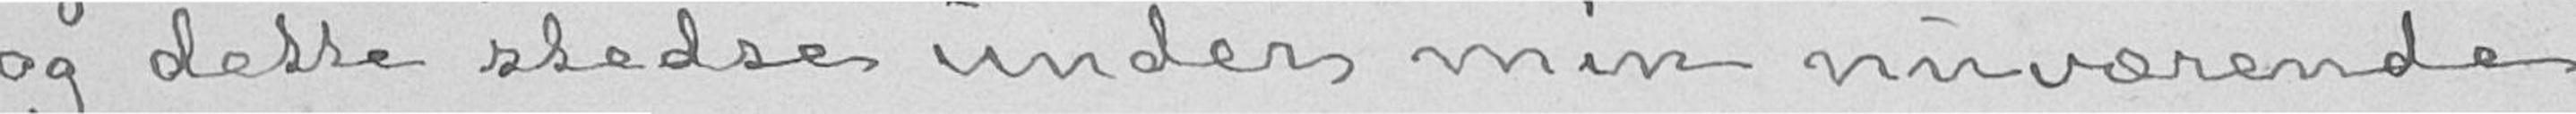og dette stedse under min nuværende
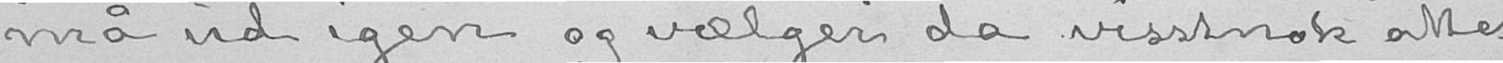må ud igen og vælger da visstnok atter
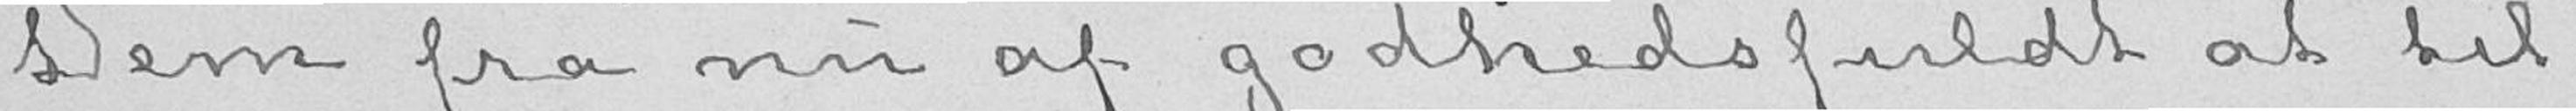Dem fra nu af godhedsfuldt at til-
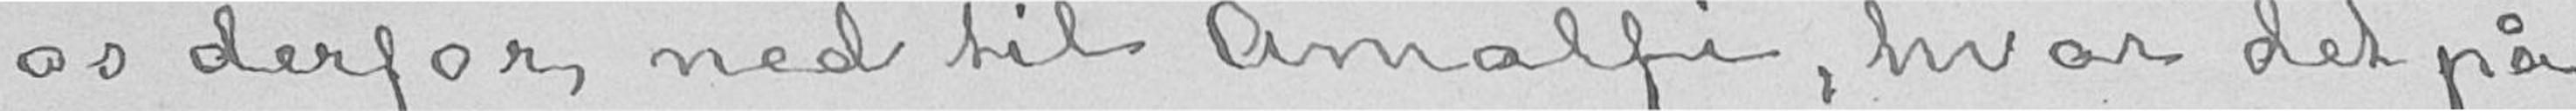os derfor ned til Amalfi, hvor det på
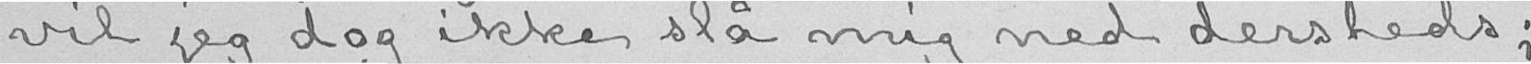vil jeg dog ikke slå mig ned dersteds;
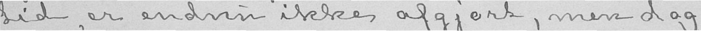tid, er endnu ikke afgjort, men dog
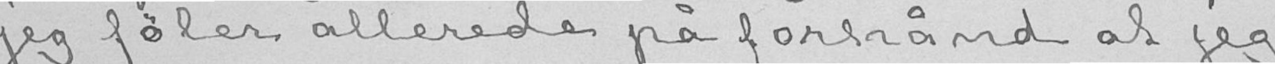jeg føler allerede på forhånd at jeg
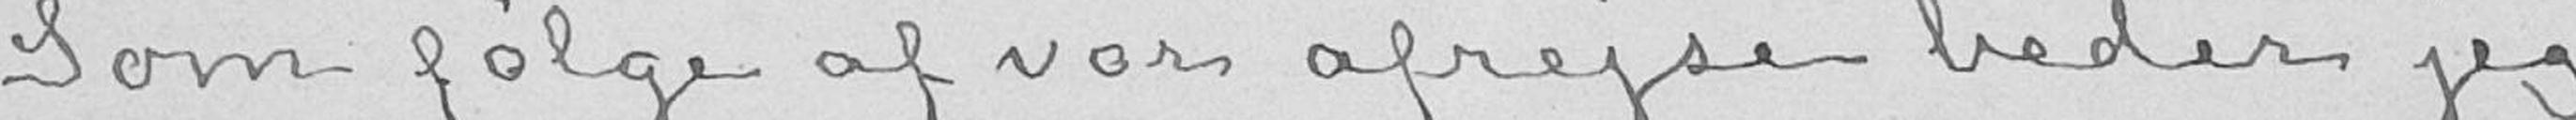Som følge af vor afrejse beder jeg
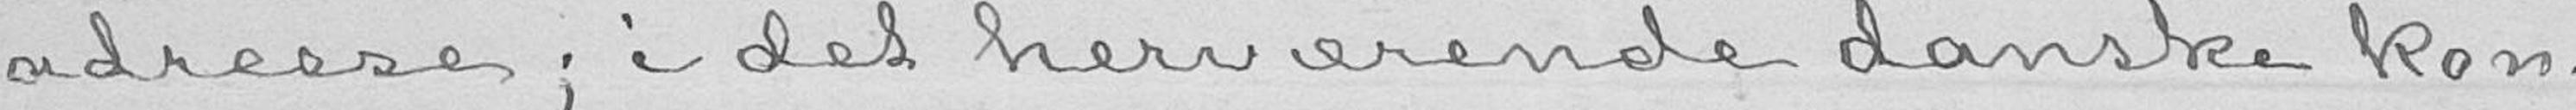adresse; i det herværende danske kon-
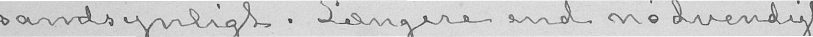sandsynligt. Længere end nødvendigt
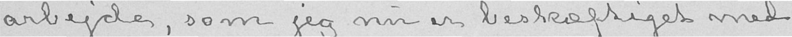arbejde, som jeg nu er beskæftiget med.
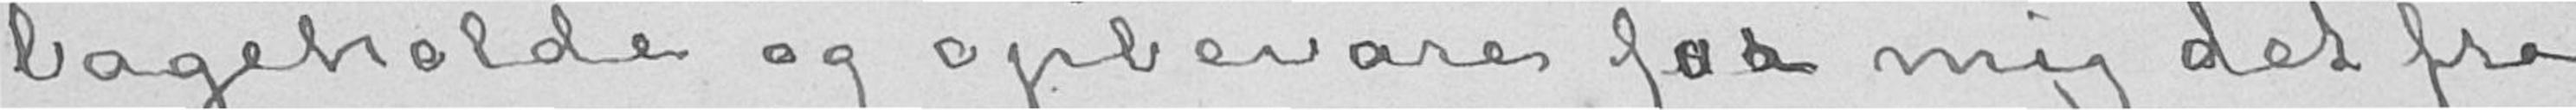bageholde og opbevare fraor mig det fra
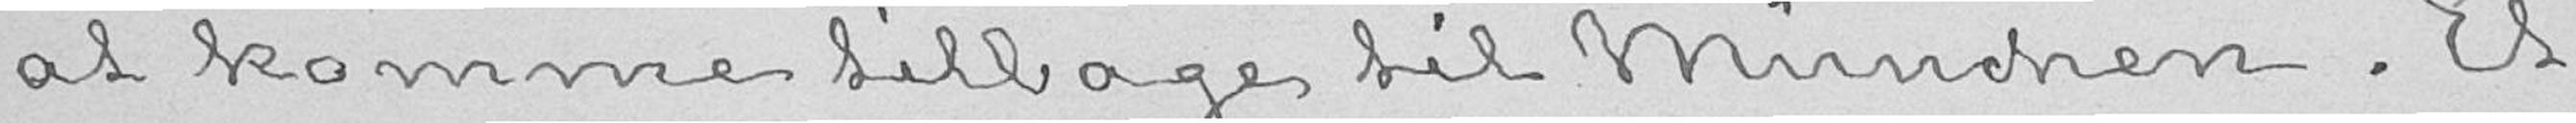at komme tilbage til München. Et
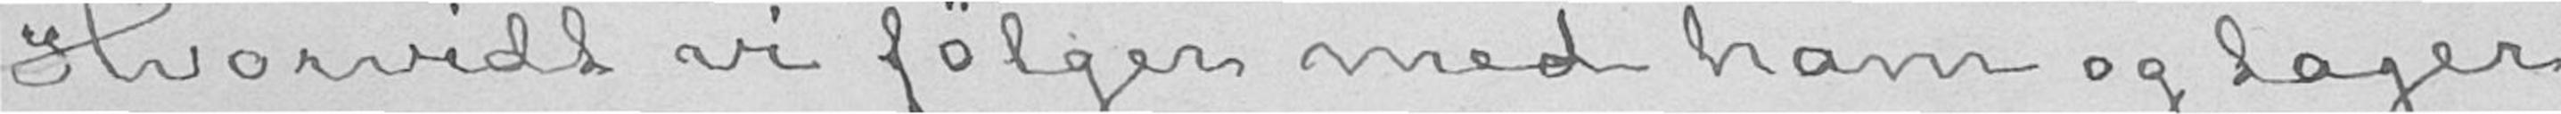Hvorvidt vi følger med ham og tager
